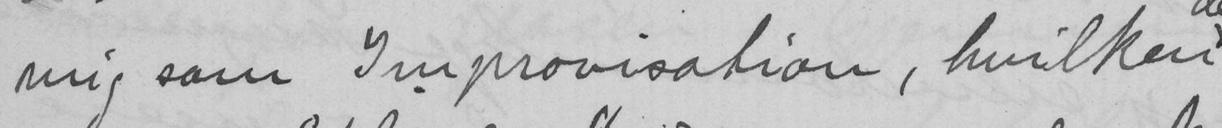mig som Improvisation, hvilken
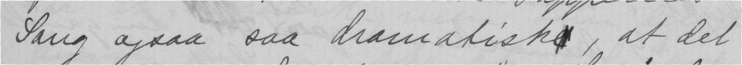Sang ogsaa saa dramatisk, at det
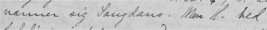nærmer sig Sangdans. Men H. ved
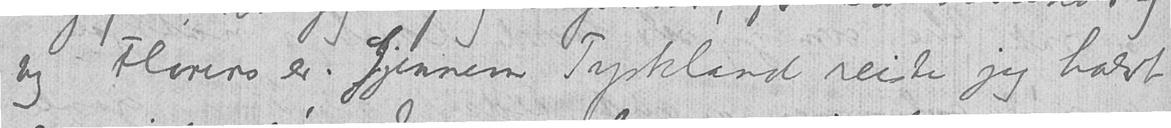by Florens er. Gjennem Tyskland reiste jeg halvt
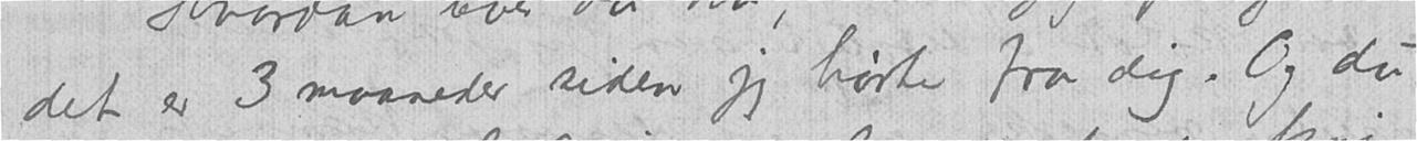det er 3 maander siden jeg hørte fra dig. Og du
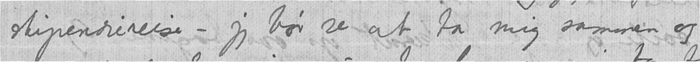stipendiereise - jeg bør se at ta mig sammen og
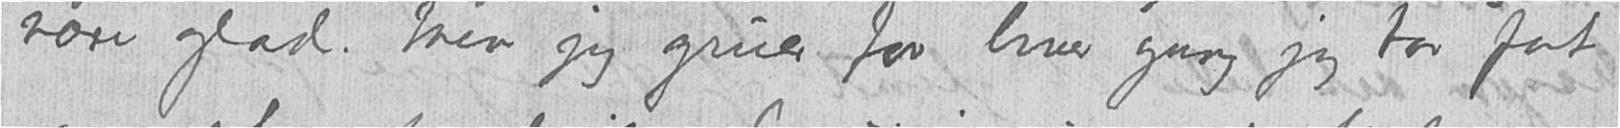være glad. Men jeg gruer for hver gang jeg tar fat
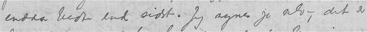endda bedre end sidst. Jeg synes jo selv, - det er
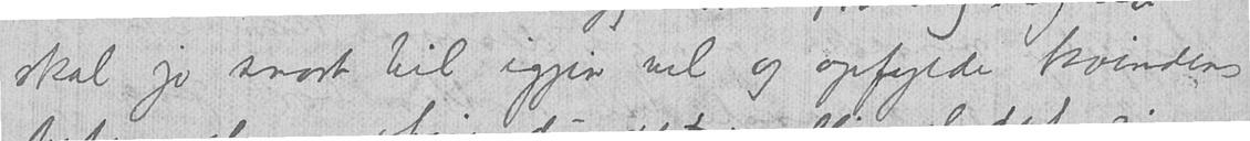skal jo snart til igjen vel og opfylde kvindens
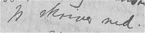jeg skriver ned.
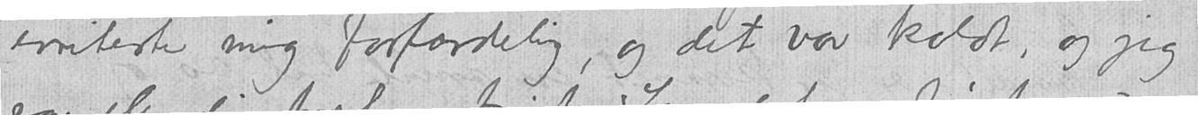irriterte mig forfærdelig, og det var koldt, og jeg
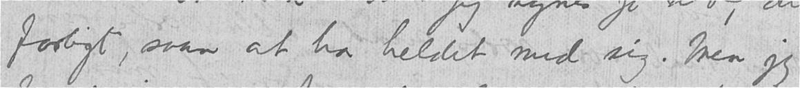farligt, saan at ha heldet med sig. Men jeg
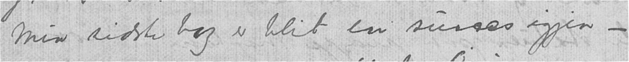Min sidste bog er blit en succes igjen -
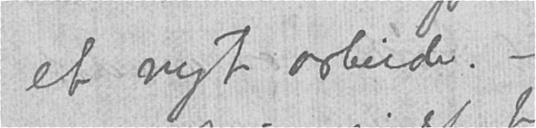et nyt arbeide. -
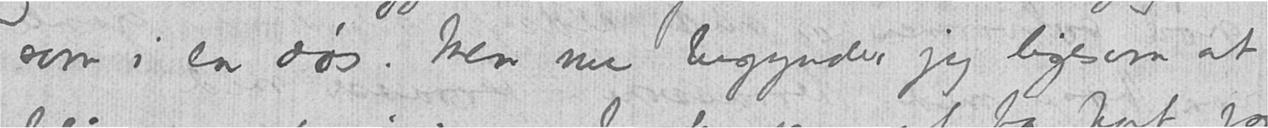som i en døs. Men nu begynder jeg ligsom at
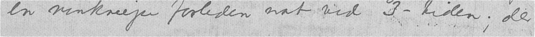en vinkneipe forleden nat ved 3-tiden; der
